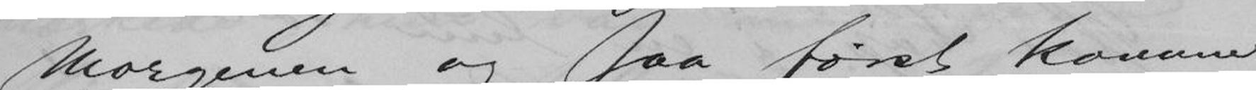Morgenen og saa først komme
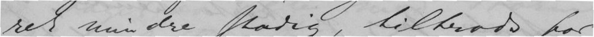ret mindre stadig, tiltrods for
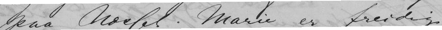paa Næsset. Marie er freidig
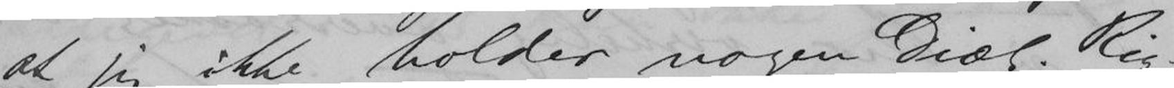at jeg ikke holder nogen Diæt. Rig-
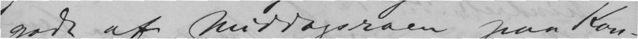godt af Middagsroen paa Kon-
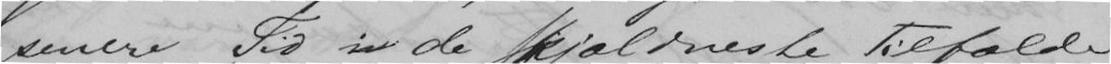senere Tid i de skjældneste Tilfælde
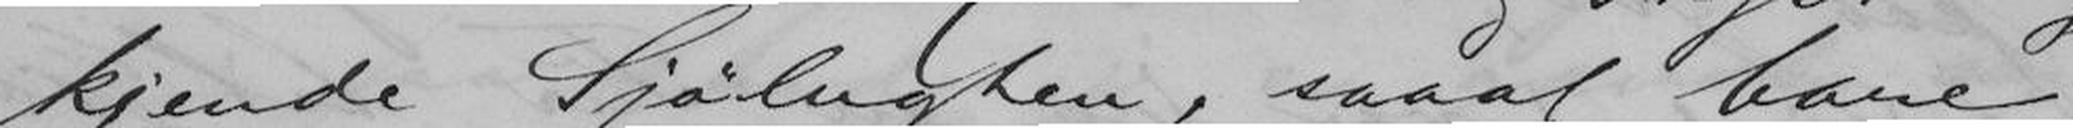kjende Sjølugten, saaat bare
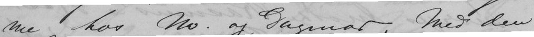me hos M. og Dagmar. Med den
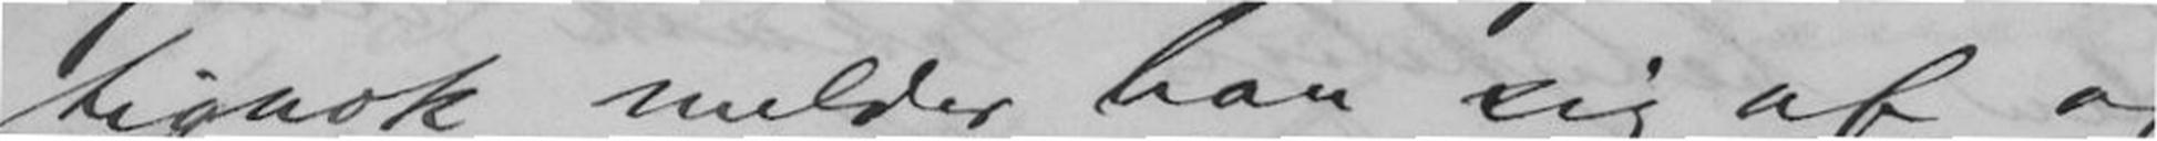tignok nulder han sig af og
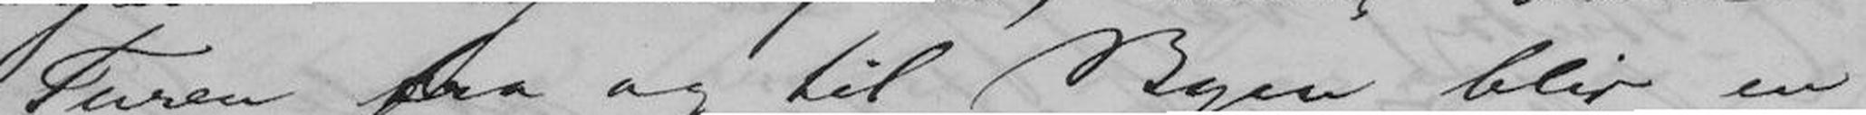Turen fra og til Byen blir en
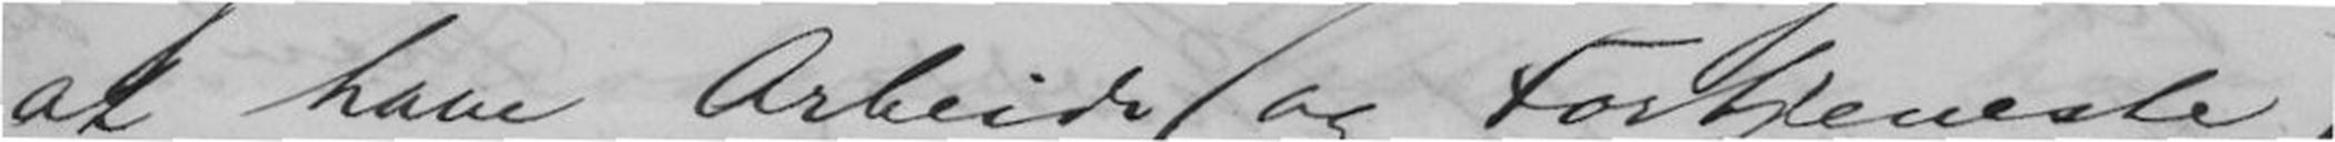at have Arbeid (og Fortjeneste)
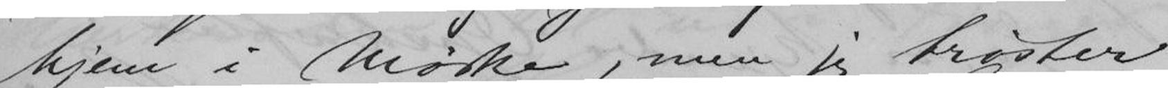hjem i Mørke, men jeg trøster
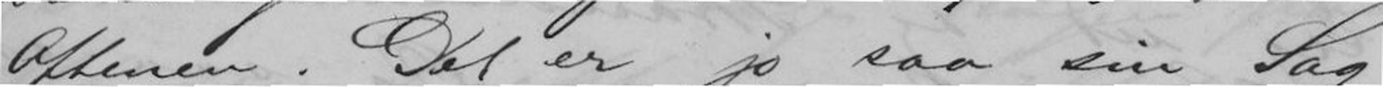Aftenen. Det er jo saa sin Sag
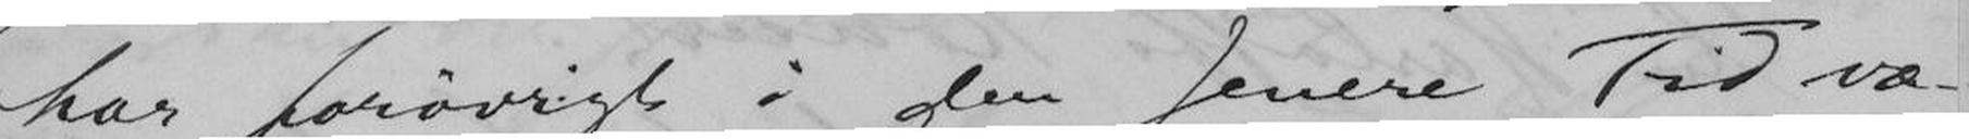har forøvrigt i den senere Tid væ-
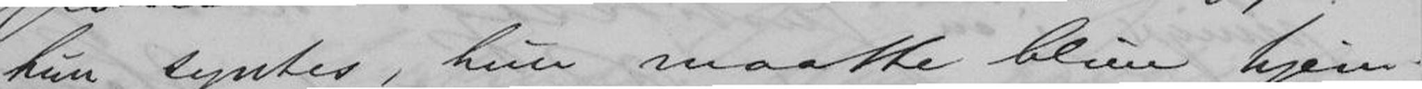hun syntes, hun maatte blive hjem-
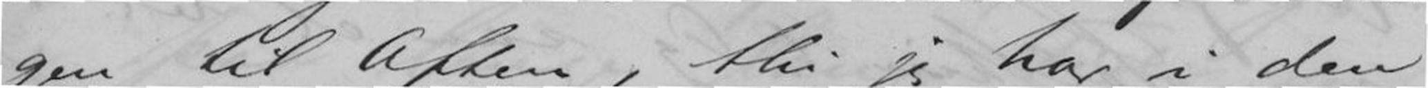gen til Aften, thi jeg har i den
